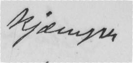kjæmper
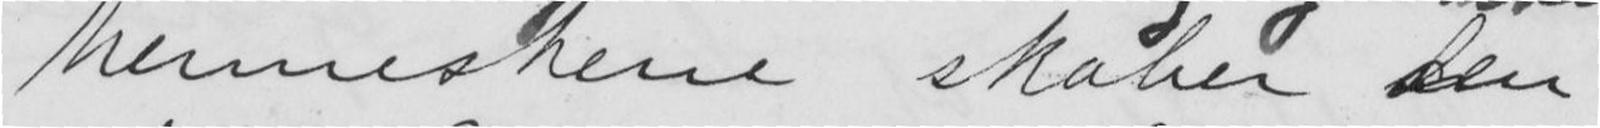Menneskene skaber den
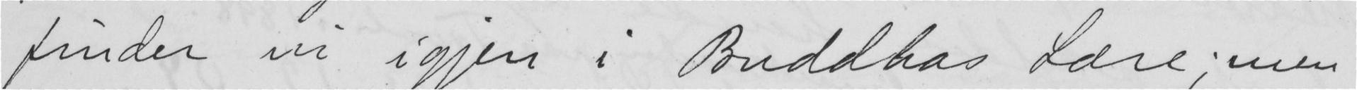finder vi igjen i Buddhas Lære; men
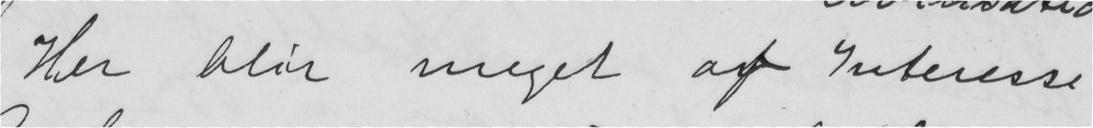Her blir meget af Interesse
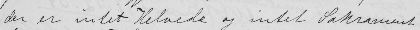der er intet Helvede og intet Sakrament
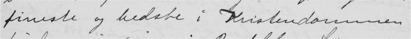fineste og bedste i Kristendommen
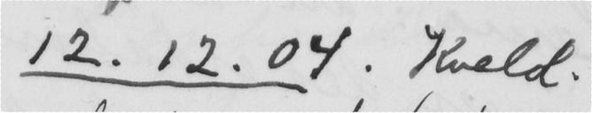12.12.04 Kveld.
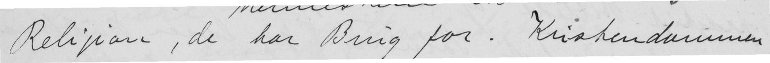Religion, de har Brug for. Kristendommen
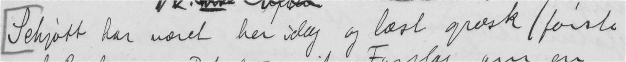Schjøtt har været her idag og læst græsk (første
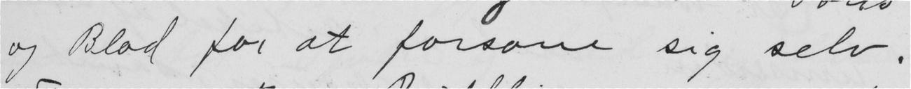og Blod for at forsone sig selv.
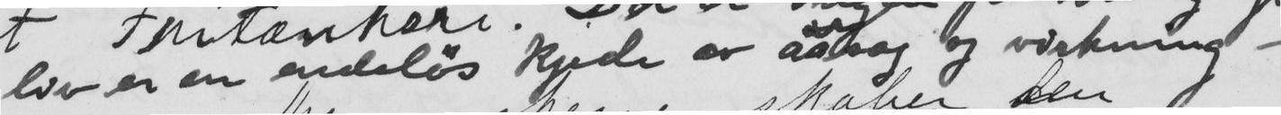liv er en endeløs kjede av aarsag og virkning -
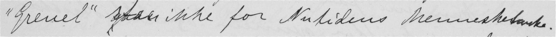"Greuel" staar ikke for Nutidens Mennesketanke.
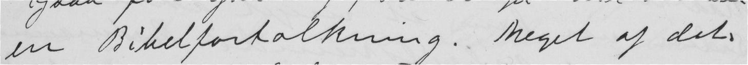en Bibelfortolkning. Meget af det
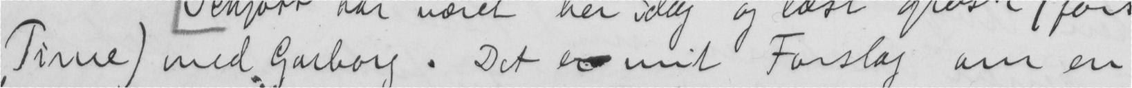Time) med Garborg. Det er mit Forslag om en
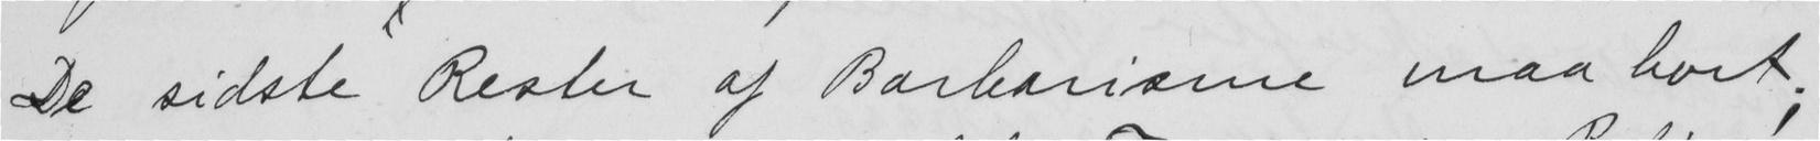De sidste Rester af Barbarisme maa bort;
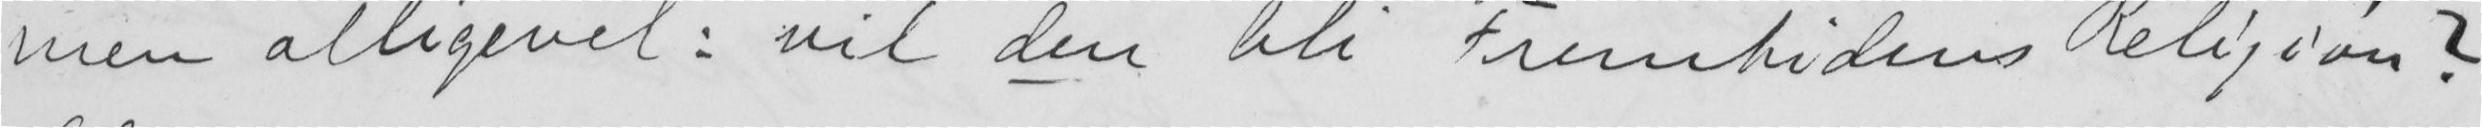men alligevel: vil den bli Fremtidens Religion?
